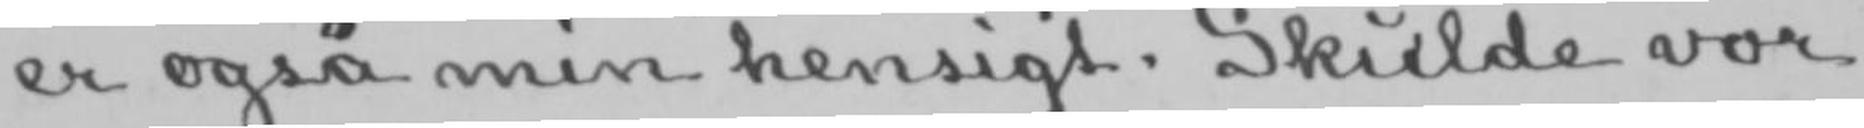er også min hensigt. Skulde vor
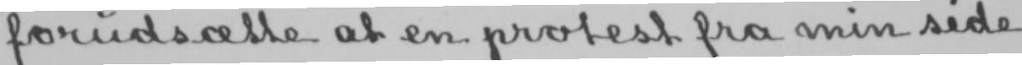forudsætte at en protest fra min side
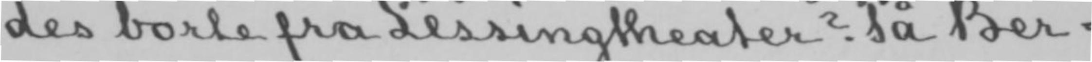des borte fra Lessingtheater? På Ber-
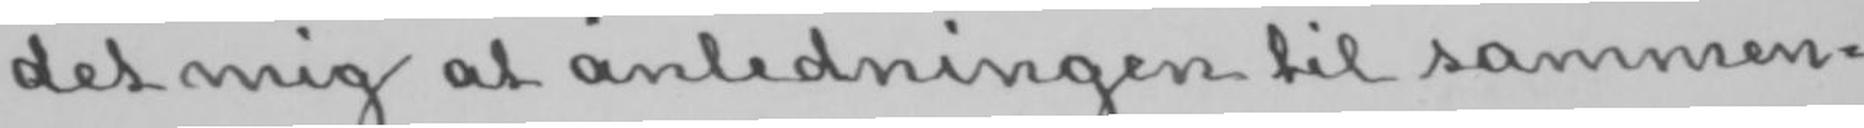det mig at anledningen til sammen-
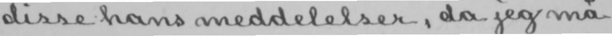disse hans meddelelser, da jeg må
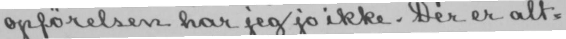opførelsen har jeg jo ikke. Der er alt-
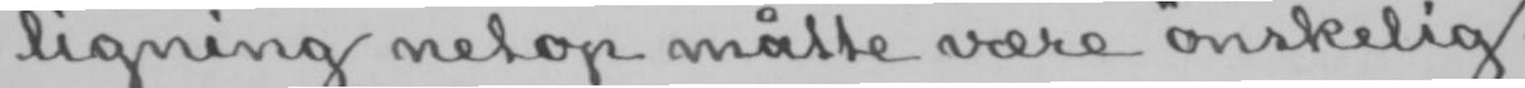ligning netop måtte være ønskelig.
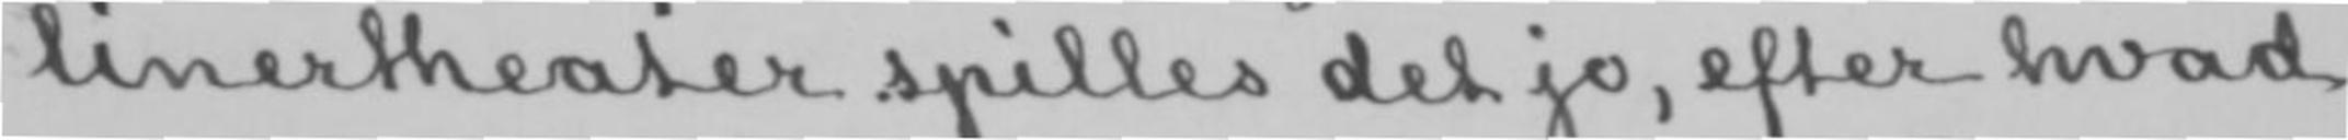linertheater spilles det jo, efter hvad
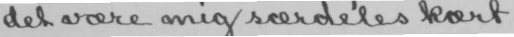det være mig særdeles kært.
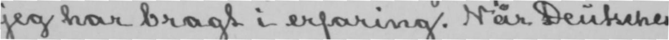jeg har bragt i erfaring. Når Deutsches
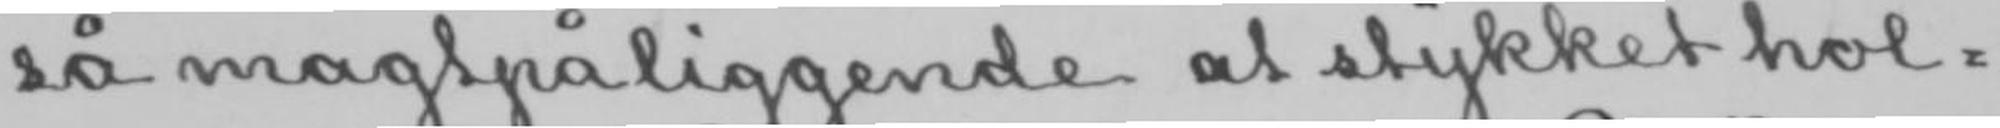så magtpåliggende at stykket hol-
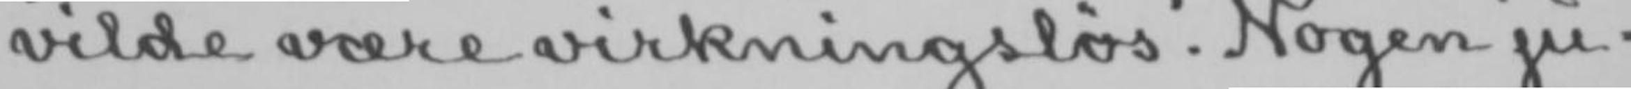vilde være virkningsløs. Nogen ju-
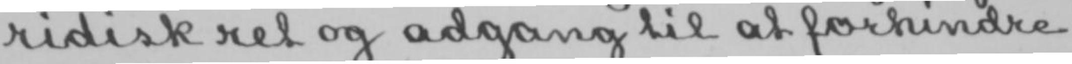ridisk ret og adgang til at forhindre
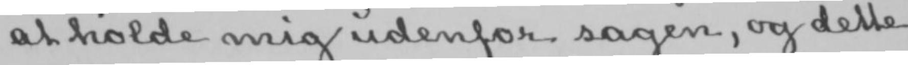at holde mig udenfor sagen, og dette
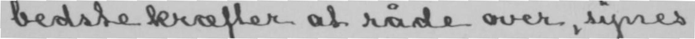bedste kræfter at råde over, synes
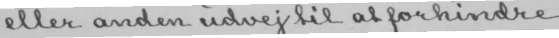eller anden udvej til at forhindre
